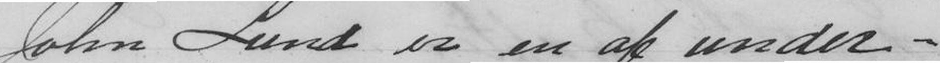John Lund er en af under-
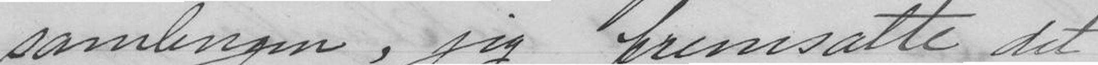samlingen, jeg fremsatte det
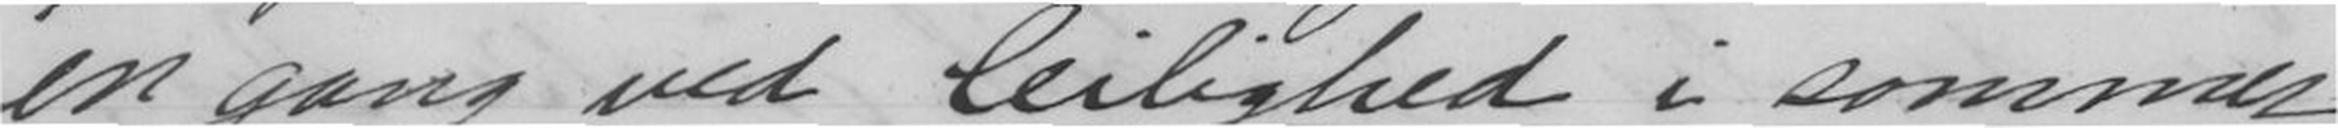engang ved leilighed i sommer
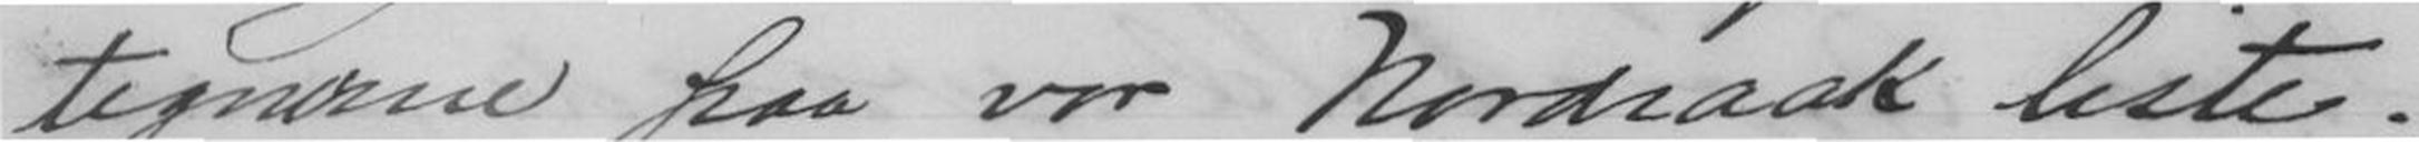tegnerne paa vor Nordraak liste.
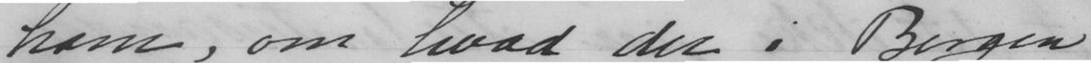ham, om hvad der i Bergen
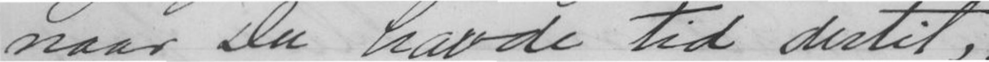naar Du havde tid dertil,
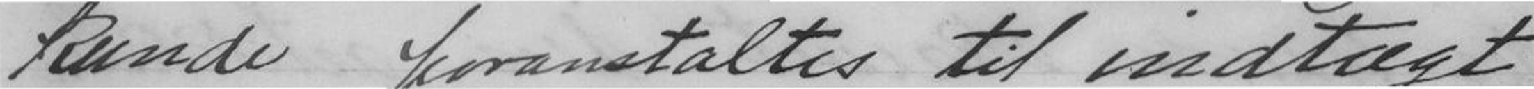kunde foranstaltes til indtægt
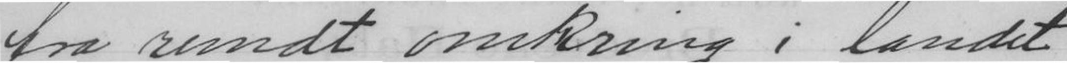fra rundt omkring i landet.
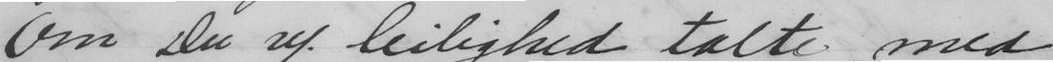Om Du v/. leilighed talte med
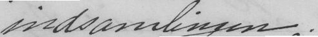indsamlingen.
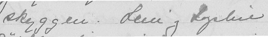skyggen. Leni og Sophie
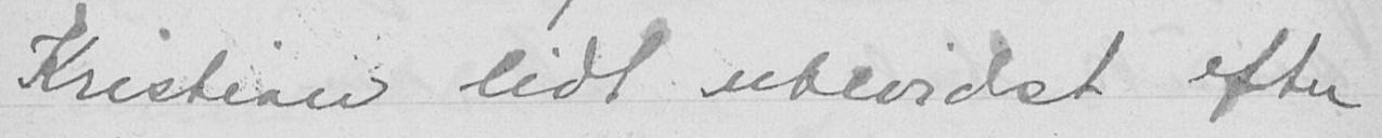Kristian lidt ubevidst efter
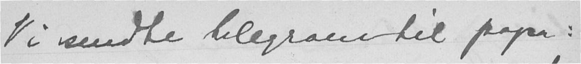Vi sendte telegram til papa:
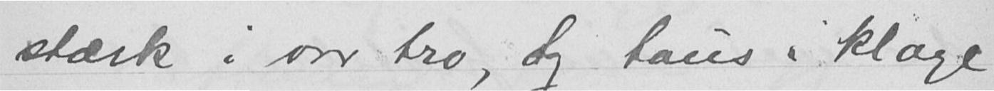stærk i vor tro, og taus i klage
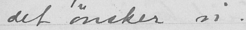det ønsker vi.
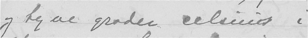og tyve grader celsius i
